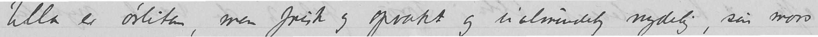Ulla er ørliten, men frisk og opvakt og ualmindelig nydelig, sin mors
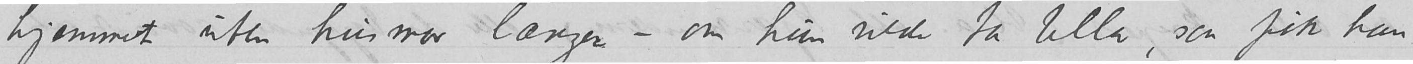hjemmet uten husmor længer – om hun vilde ta Ulla, saa fik han
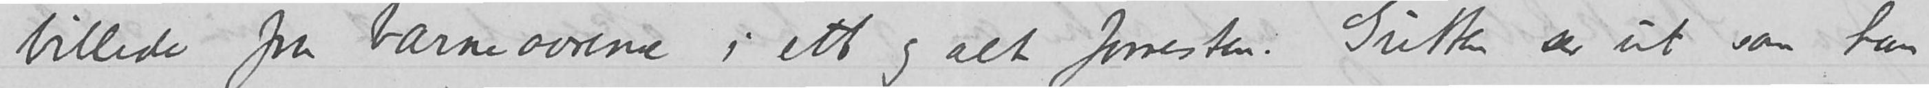billede fra barneaarene i ett og alt forresten. Gutten ser ut som han
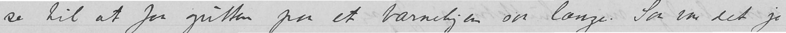se til at faa gutten paa et barnehjem saa længe. Saa var det jo
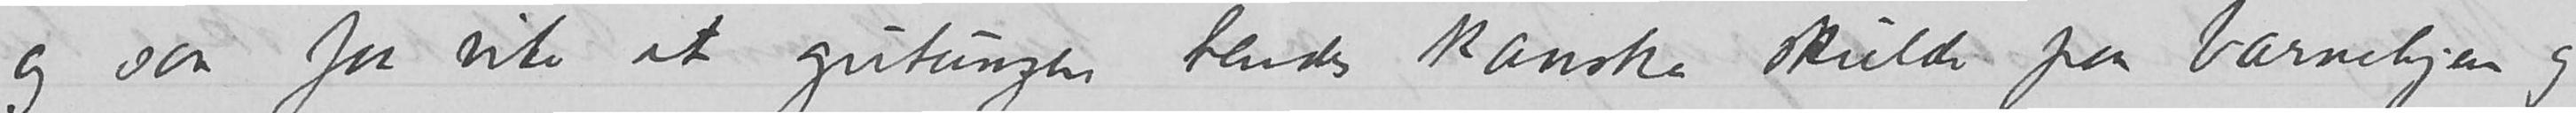og saa faa vite at gutungen hendes kanske skulde paa barnehjem og
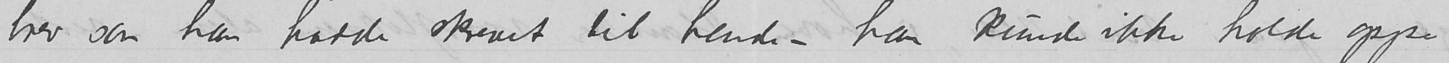brev som han hadde skrevet til hende – han kunde ikke holde oppe
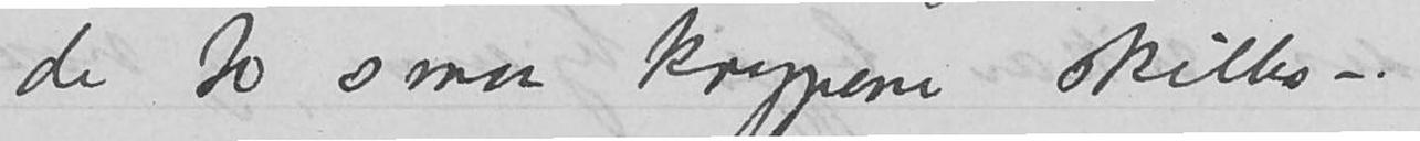de to smaa krypene skilles –.
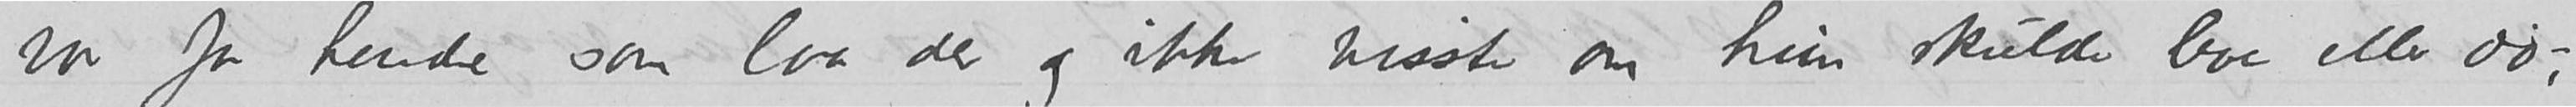var for hende som laa der og ikke visste om hun skulde leve eller dø –,
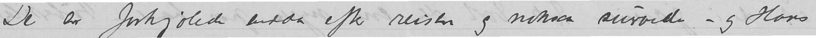De er forkjølede endda efter reisen og noksaa surrede – og Hans
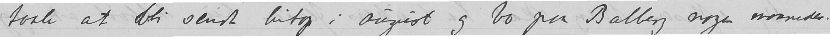taale at bli sendt hitop i august og bo paa Balberg nogen maaneder.
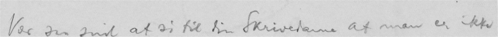Vær saa snil at si til din Skrivedame at man er ikke
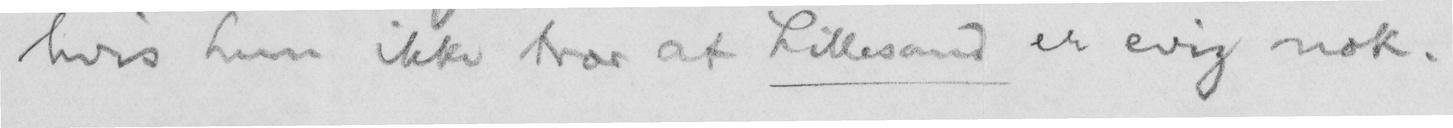hvis hun ikke tror at Lillesand er evig nok.
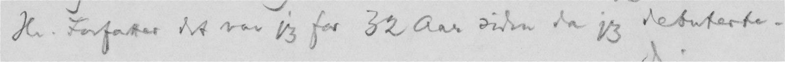Hr. Forfatter det var jeg for 32 Aar siden da jeg debuterte.
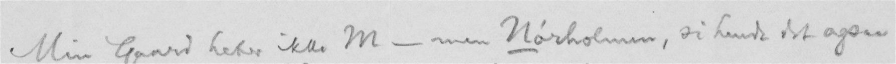Min Gaard heter ikke M - men Nørholmen, si hende det ogsaa
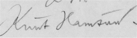Knut Hamsun.
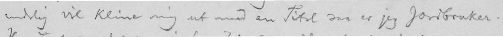endelig vil kline mig ut med en Titel saa er jeg Jordbruker.
